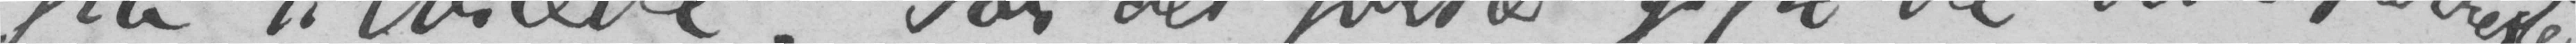fra tiltræde. For det første gifte de allerfærreste
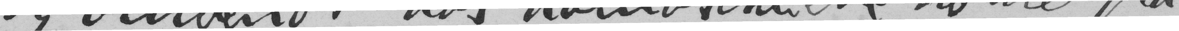og omvendt hos homosexuelle døttre fra
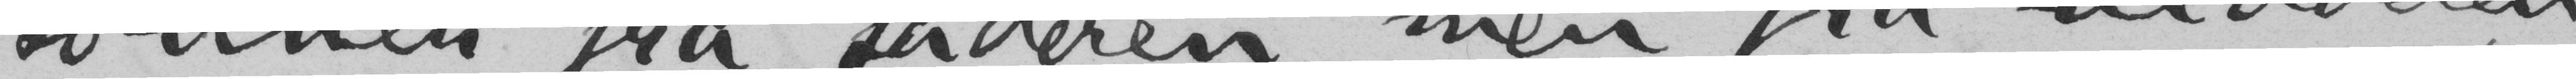sønner fra faderen, men fra moderen,
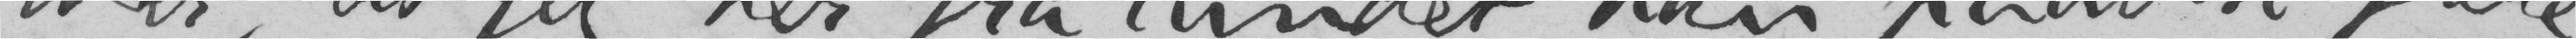mer, at jeg her fra landet kan paavise flere
